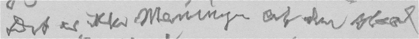Det er ikke Meningen at du skal
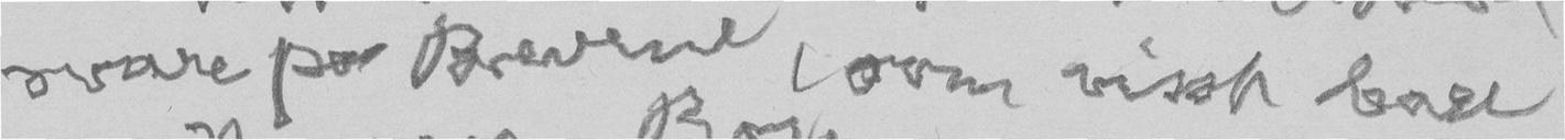svare paa Brevene, som visst bare
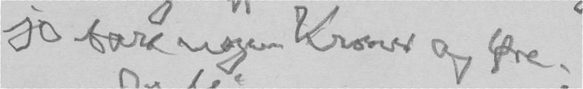jo bare nogen Kroner og Øre.
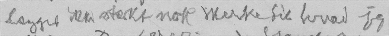lægger ikke stærkt nok Merke til hvad jeg
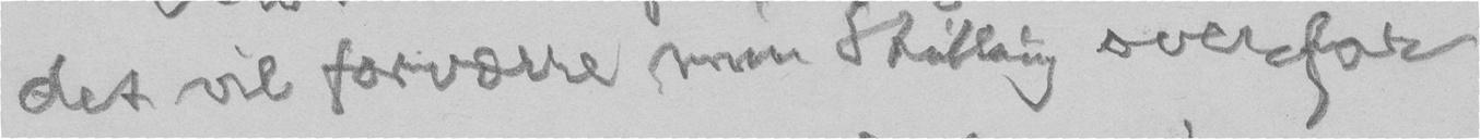det vil forværre min Stilling overfor
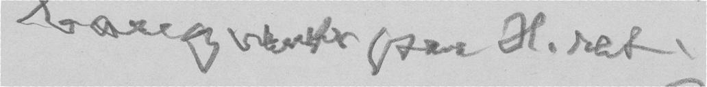bare og venter paa H.ret,
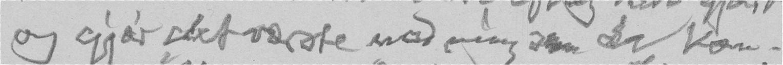og gjør det værste med mig som de kan.
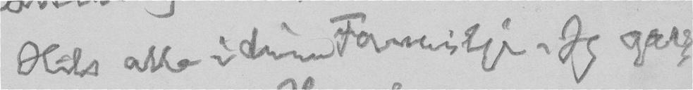Hils alle i dine Familie. Jeg gaar
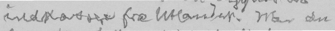indkassere fra Utlandet. Men du
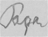Papa
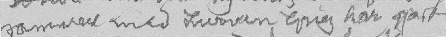sammen med Lurven Grieg har gjort
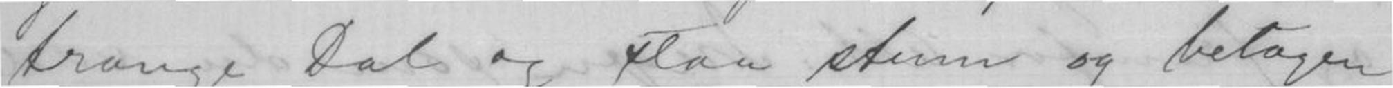trange Dal og staa stum og betagen
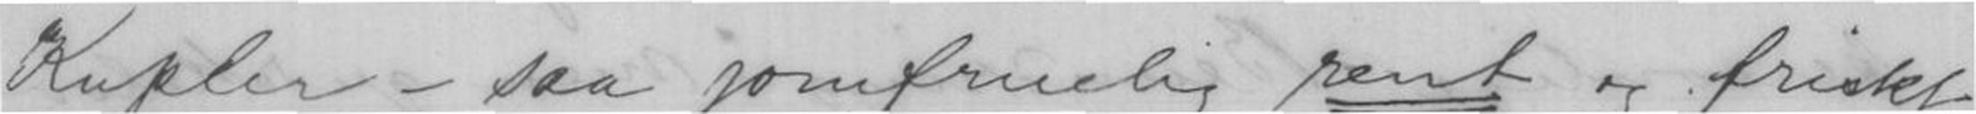Kupler - saa jomfruelig rent og friskt
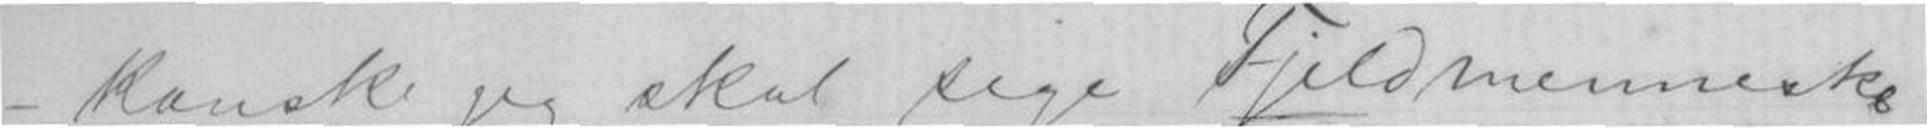- kanske jeg skal sige Fjeldmenneske
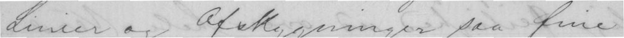Linier og Afskygninger saa fine
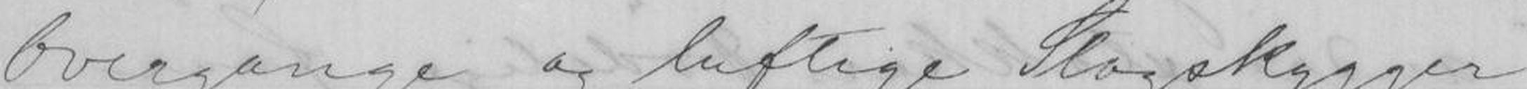Overgange og luftige Skogskygger
